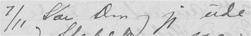7/11 Var Don og jeg ude
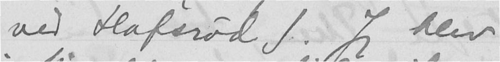ved Hafsrød). Jeg blev
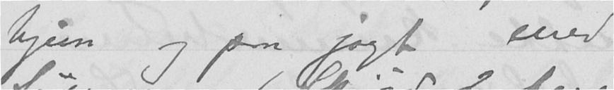hjem og paa jagt med
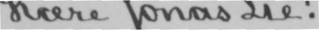Kære Jonas Lie!
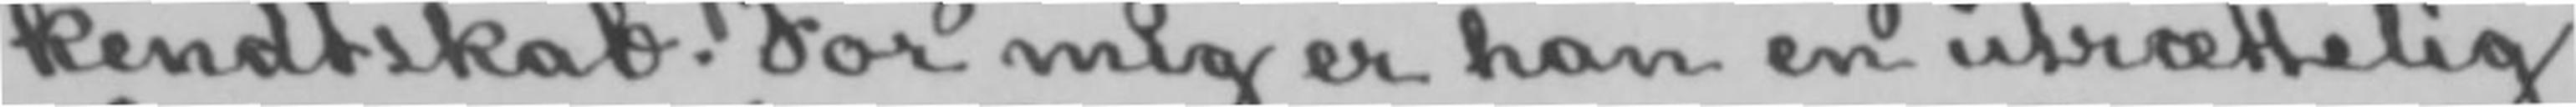kendtskab. For mig er han en utrættelig
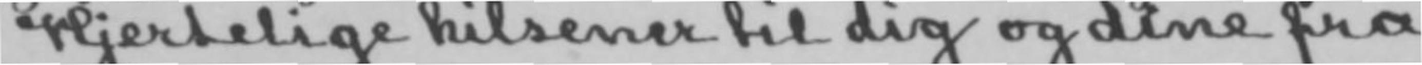Hjertelige hilsener til dig og dine fra
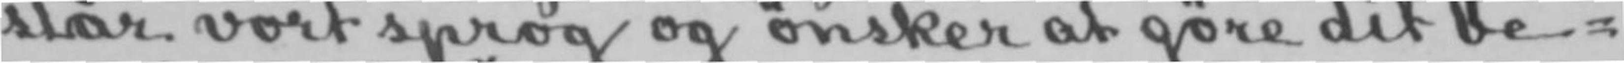står vort sprog og ønsker at gøre dit be-
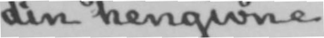din hengivne
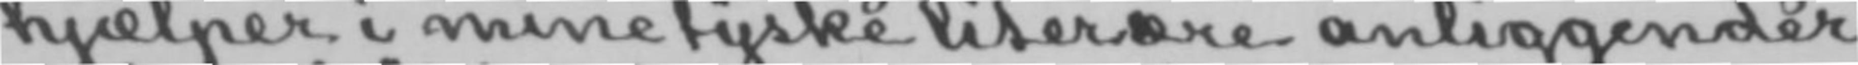hjælper i mine tyske literære anliggender
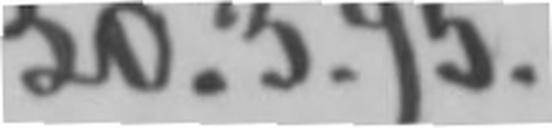30.3.95.
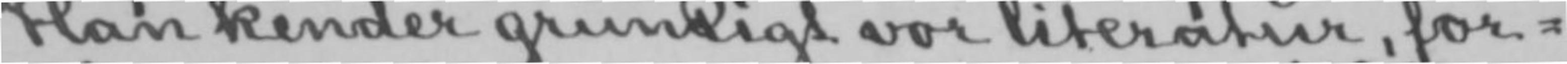Han kender grundigt vor literatur, for-
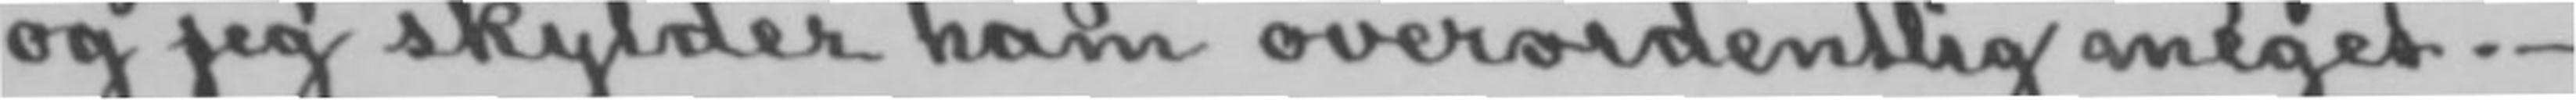og jeg skylder ham overordentlig meget.-
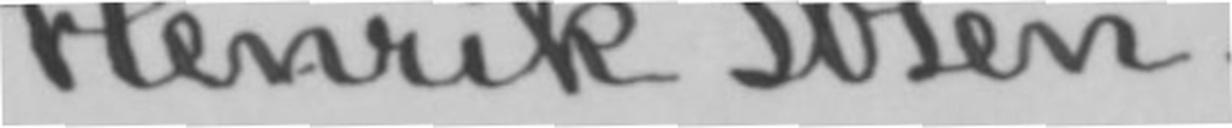Henrik Ibsen.
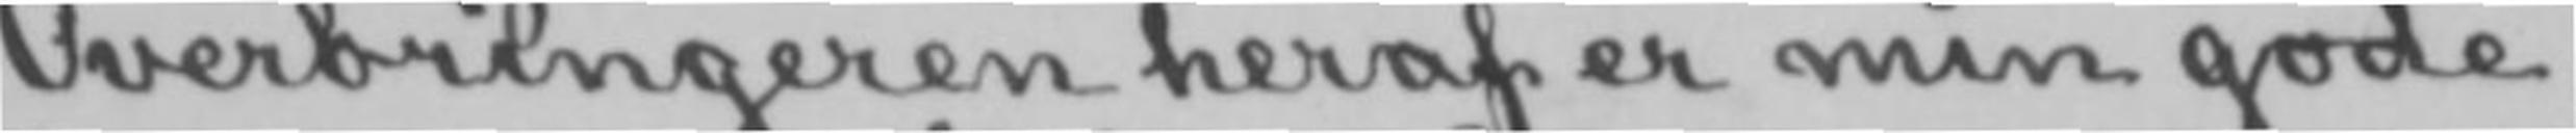Overbringeren heraf er min gode,
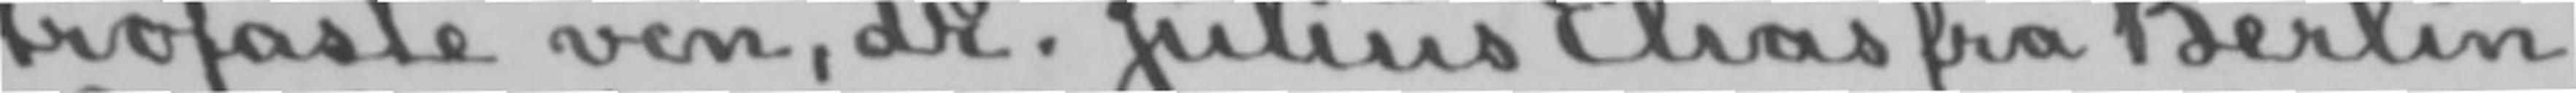trofaste ven, dr. Julius Elias fra Berlin.
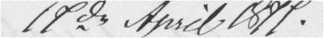17de April 1877.
